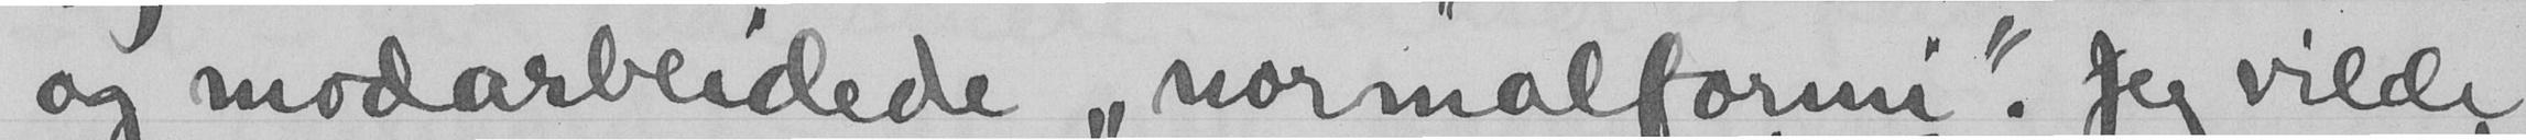og modarbeidede "normalformi". Jeg vilde
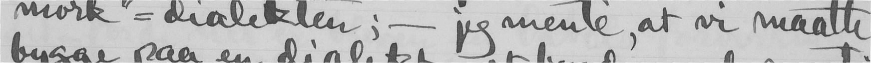mark-dialekten ; - jeg mente, at vi maate
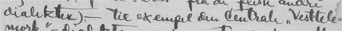dealikter) - til exempel den centrale "Vest-tele-
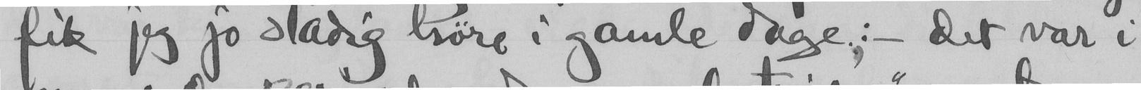fik jeg jo stadig høre i gamle dage, : - det var i
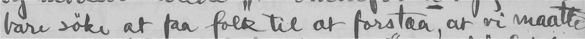bare søke at faa folk til at forstaa, at vi maatte
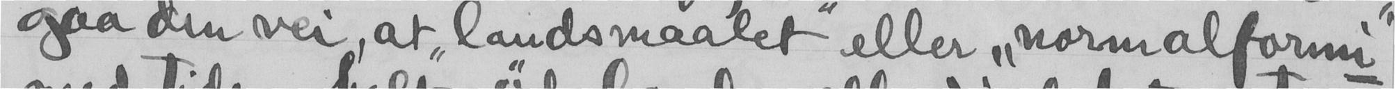gaa den vei, at "landsmaalet" eller "normalformi"
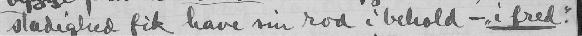stadighed fik have sin rod i behold - "ifred".
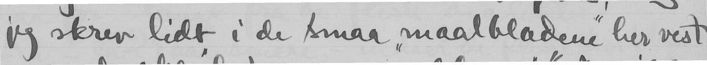jeg skrev lidt i de smaa "maalbladene" her vest
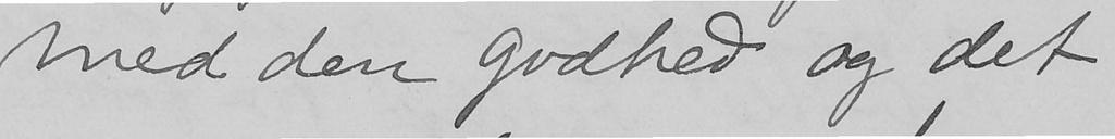med den godhed og det
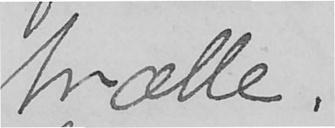trælle.
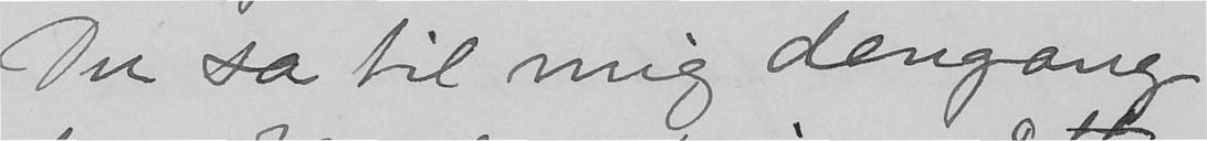Du sa til mig dengang
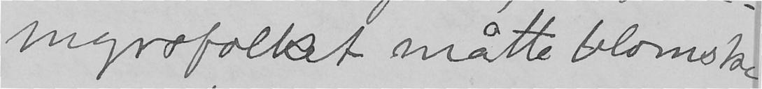myrsfolket måtte blomstre
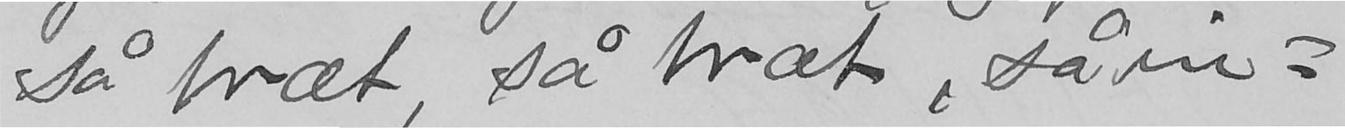så træt, så træt, så in-
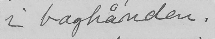i baghånden.
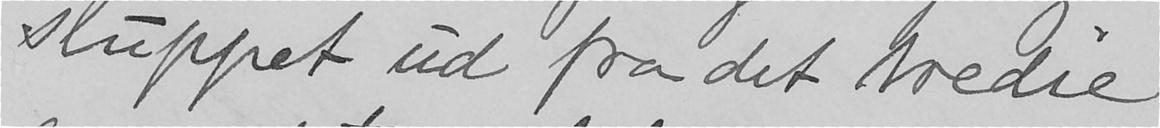sluppet ud fra det tredie
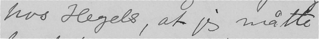hos Hegels, at jeg måtte
